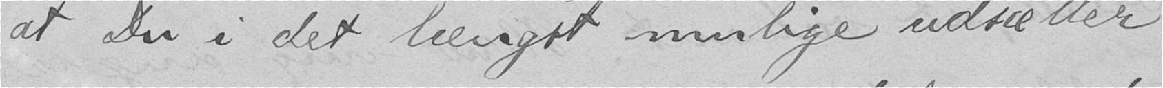at Du i det længst mulige udsætter
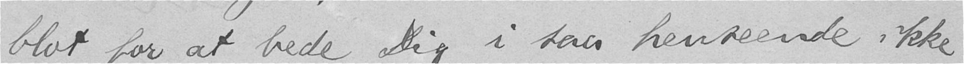blot for at bede Dig i saa henseende ikke
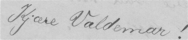Kjære Valdemar!
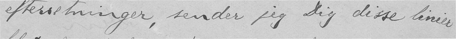efterretninger, sender jeg Dig disse linier
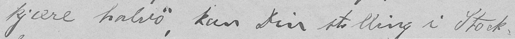kjære halvø, kan Din stilling i Stock-
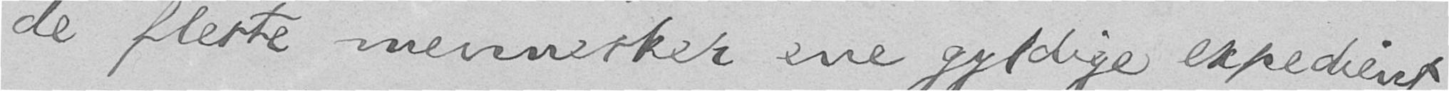de fleste mennesker ene gyldige expedient:
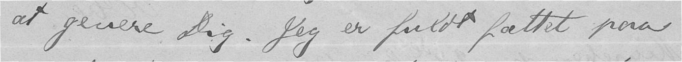at genere Dig. Jeg er fuldt fattet paa
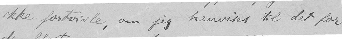ikke fortvivle, om jeg henvises til det for
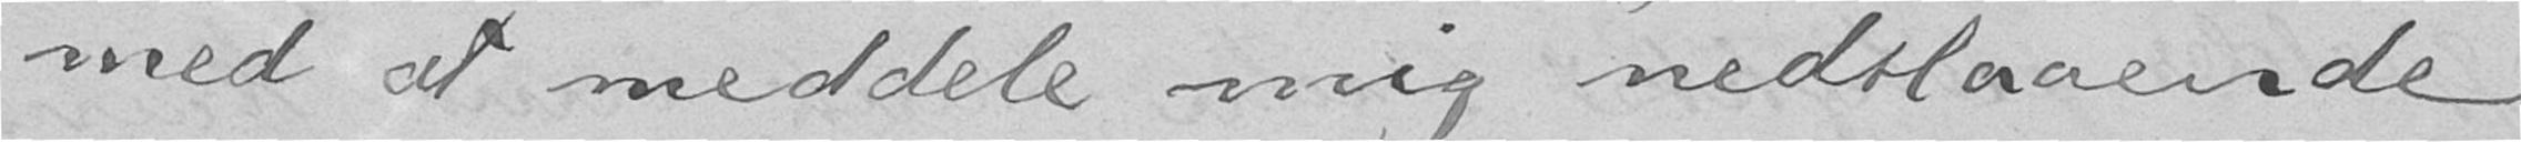med at meddele mig nedslaaende
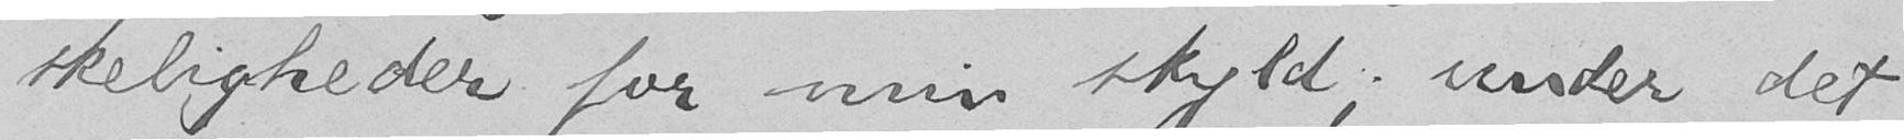skeligheder for min skyld; under det
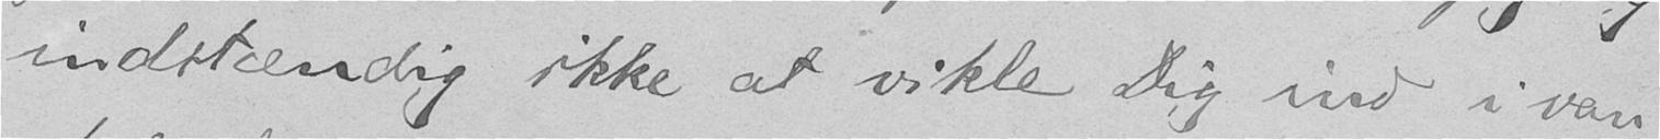indstændig ikke at vikle Dig ind i van-
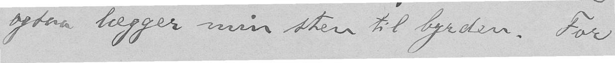ogsaa lægger min sten til byrden. For
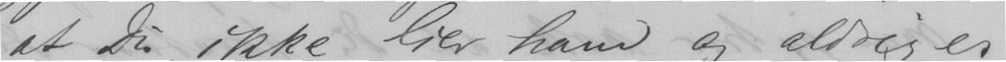at Du ikke lier ham og aldrig er
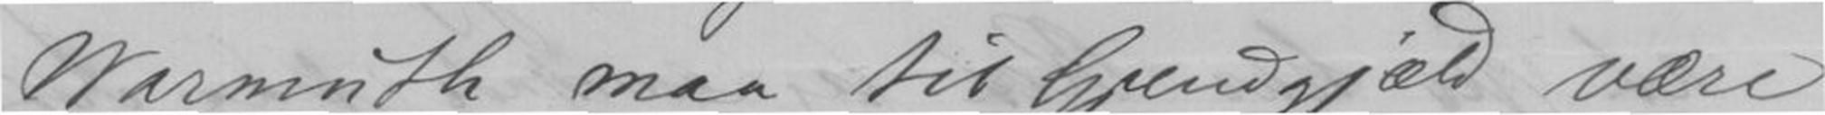Warmuth maa til Gjengjæld være
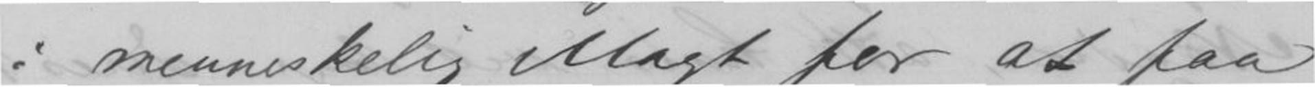i menneskelig Magt for at faa
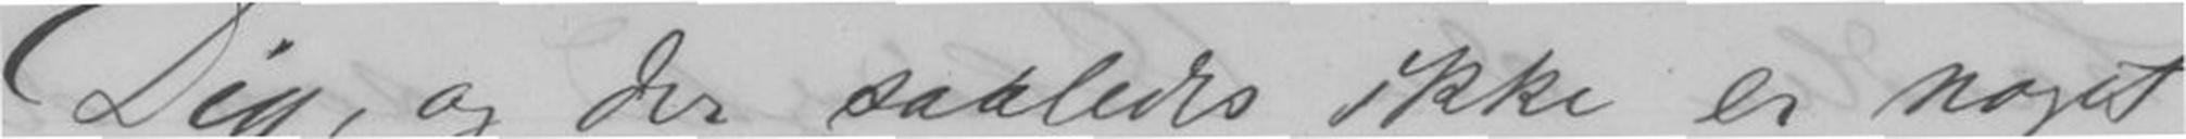Dig, og der saaledes ikke er noget
